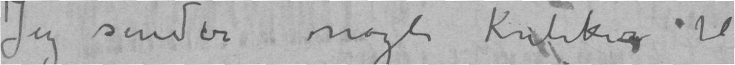Jeg sender nogle Kritikker til
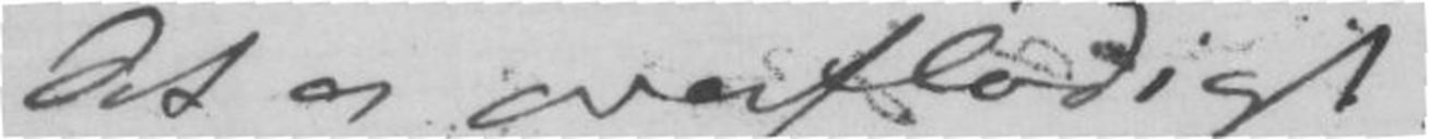det er overflødigt.
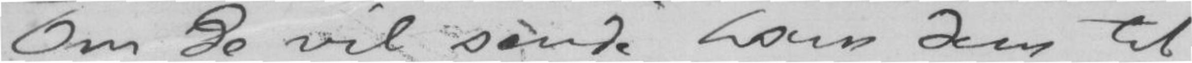Om De vil sende ham dem til
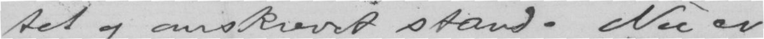de som Grieg vil have dem.
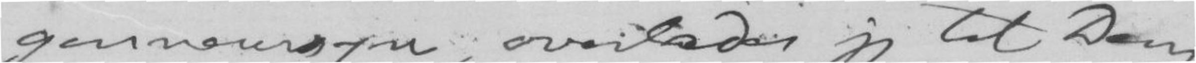gennemsyn, overlader jeg til Dem
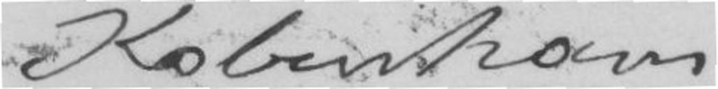København
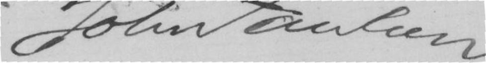John Paulsen.
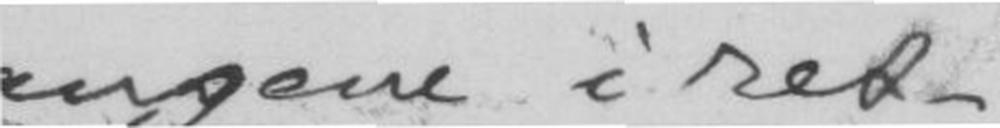tet og omskrevet stand. Nu er
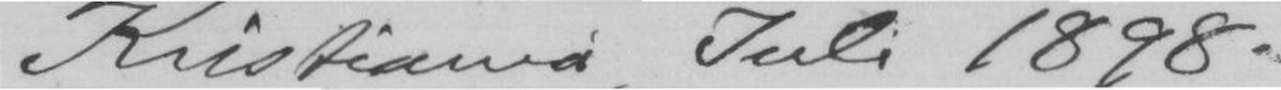Kristiania, Juli 1898.
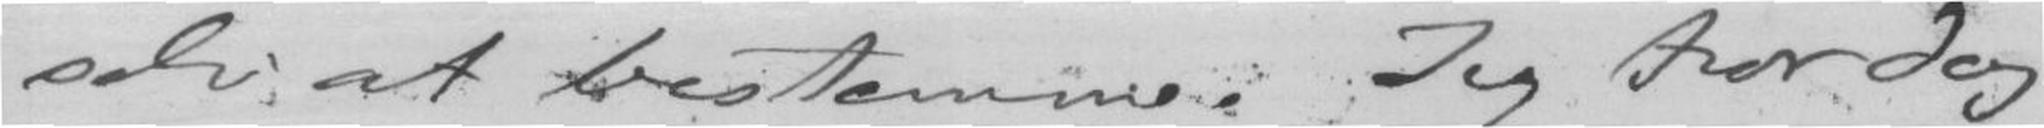selv at bestemme. Jeg trot dog
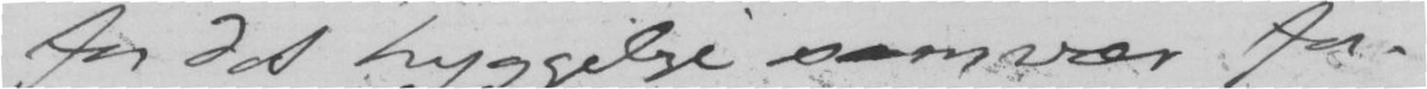for det hyggelige samvær for-
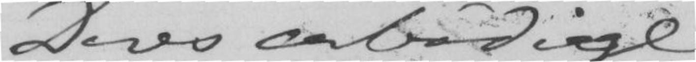Deres ærbødige
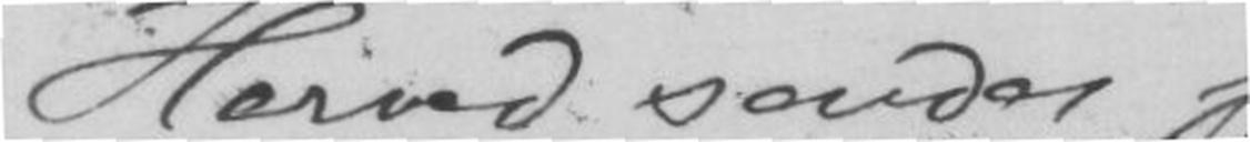Hermed sender jeg sangene i ret-
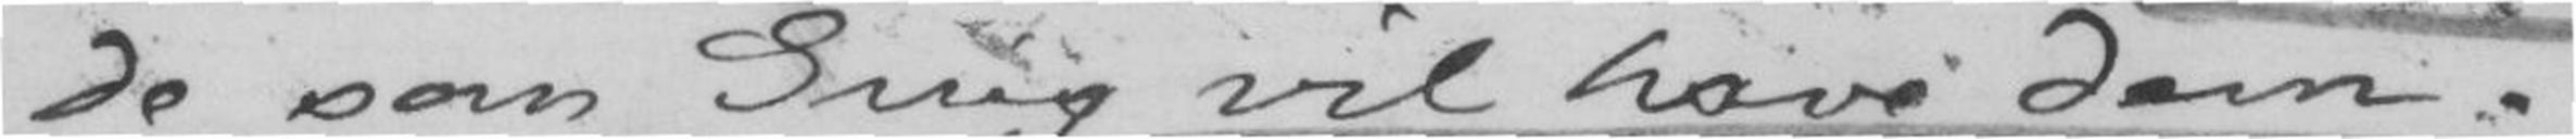Understreking ikke gjort av J.P.
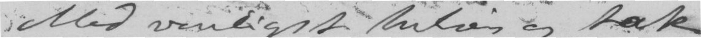Med venligst hilsen og tak
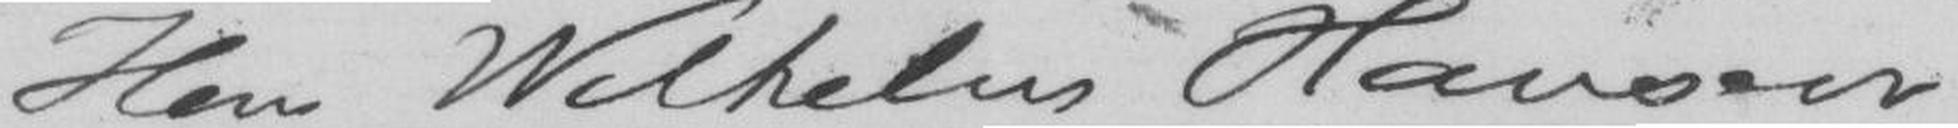Herr Wilhelm Hansen
